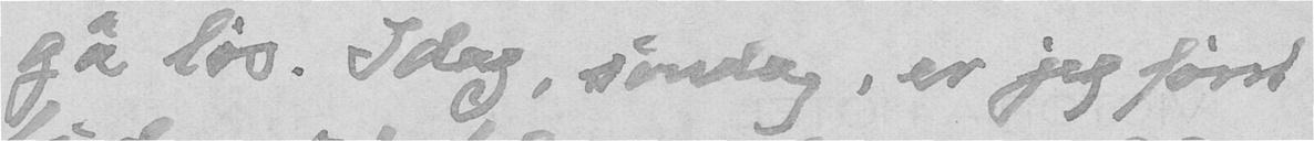gå løs. Idag, søndag, er jeg først
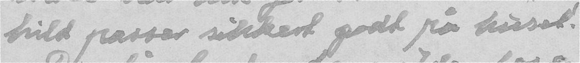hild passer sikkert godt på huset!
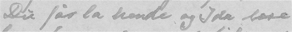Du får la hende, og Ida lese
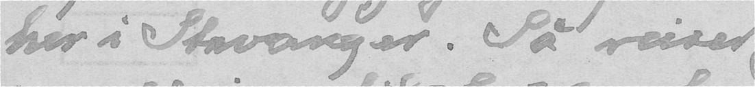her i Stavanger. Så reiser jeg
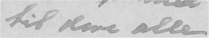til dere alle
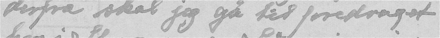derfra skal jeg gå til foredraget
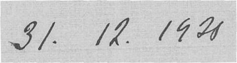31.12.1921
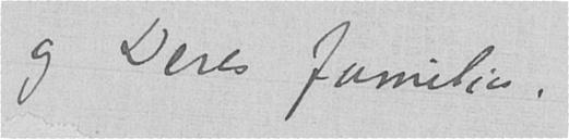og Deres familier,
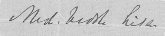Med bedste hilsen
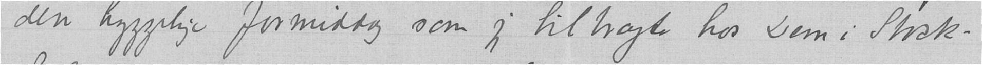den hyggelige formiddag som jeg tilbragte hos Dem i Stock-
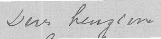Deres hengivne
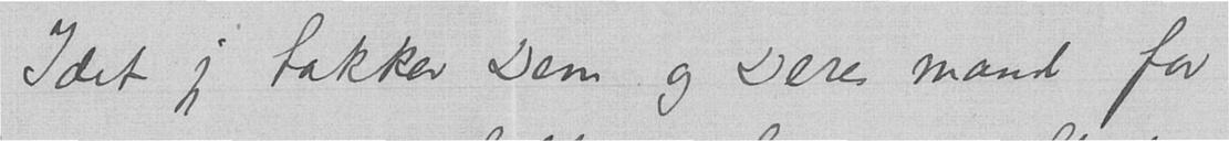Idet jeg takker Dem og Deres mand for
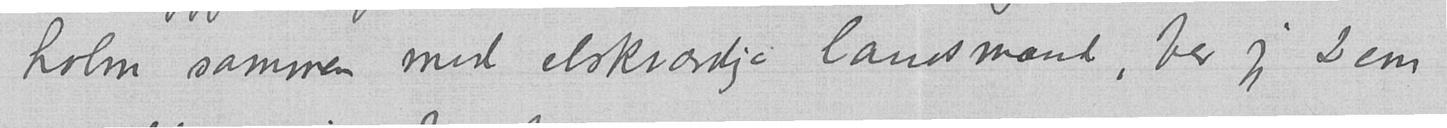holm sammen med elskværdige landsmænd, ber jeg Dem
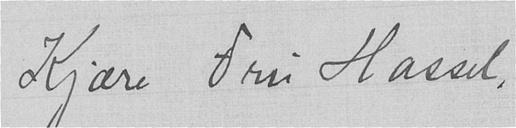Kjære Fru Hassel,
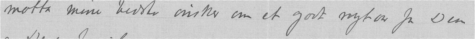motta mine bedste ønsker om et godt nytaar for Dem
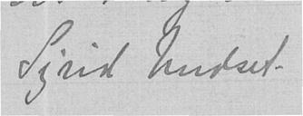Sigrid Undset.
